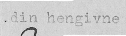din hengivne
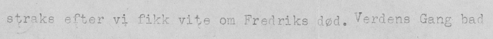straks efter vi fikk vite om Fredriks død. Verdens Gang bad
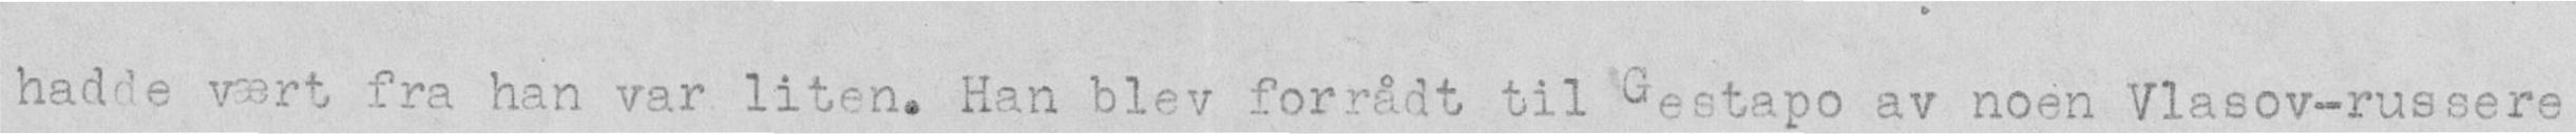hadde vært fra han var liten. Han blev forrådt til Gestapo av noen Vlasov-russere
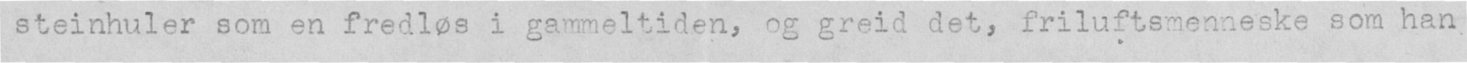steinhuler som en fredløs i gammeltiden, og greid det, friluftsmenneske som han
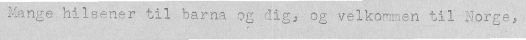Mange hilsener til barna og dig, og velkommen til Norge,
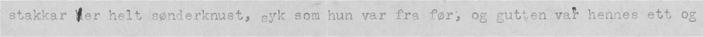stakkar her helt sønderknust, syk som hun var fra før, og gutten var hennes ett og
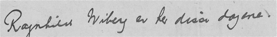Ragnhild Wiberg er te disse dagene.
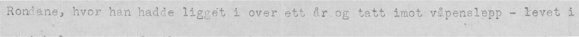Rondane, hvor han hadde ligget i over ett år og tatt imot våpenslepp - levet i
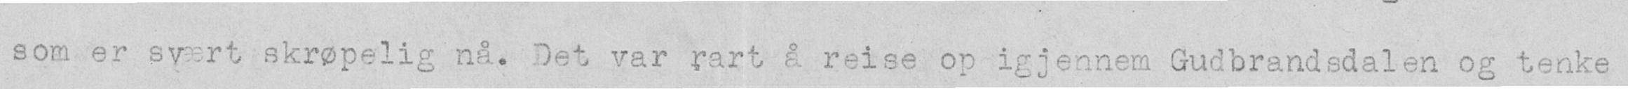som er svert skrøpelig nå. Det var rart å reise op igjennem Gudbrandsdalen og tenke
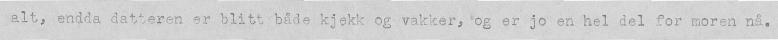alt, endda datteren er blitt både kjekk og vakker, og er jo en hel del for moren nå.
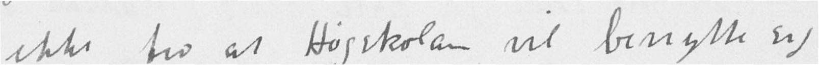ikke tro at Høgskolan vil benytte sig
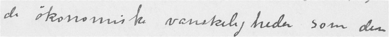de økonomiske vanskeligheder som den
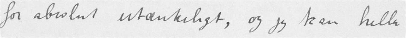for absolut utænkeligt, og jeg kan heller
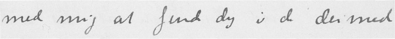med mig at finde dig i de dermed
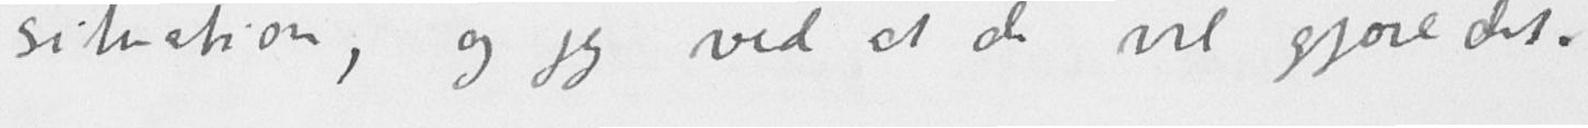situation, og jeg ved at de vil gjøre det.
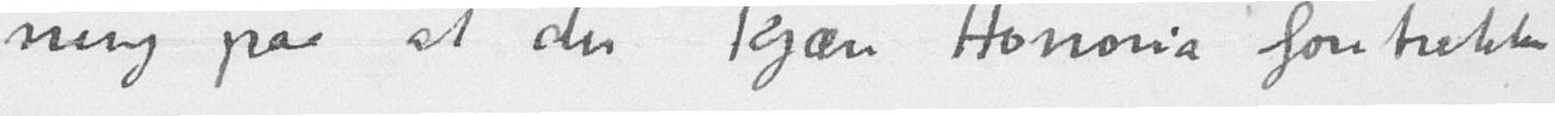ning paa at du kjære Honoria foretrækker
